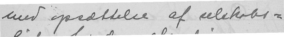med opsættelse af selskabs-
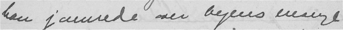han jamrede over byens mange
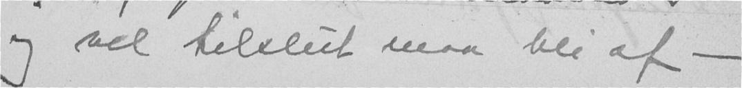og vel tilslut maa bli af -
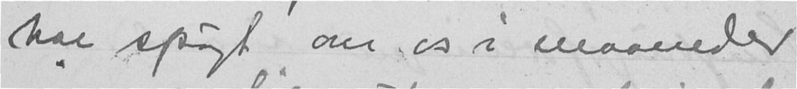har spøgt om os i maaneder
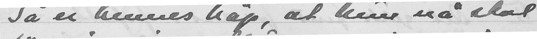Så er hennes håp, at hun nå skal
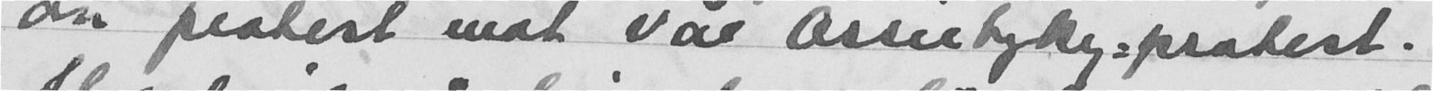om protest mot vår Ossietzky-protest.
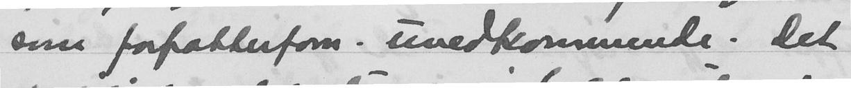som forfatterforn. uvedkommende. Det
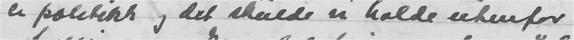er politikk og det skulde vi holde utenfor
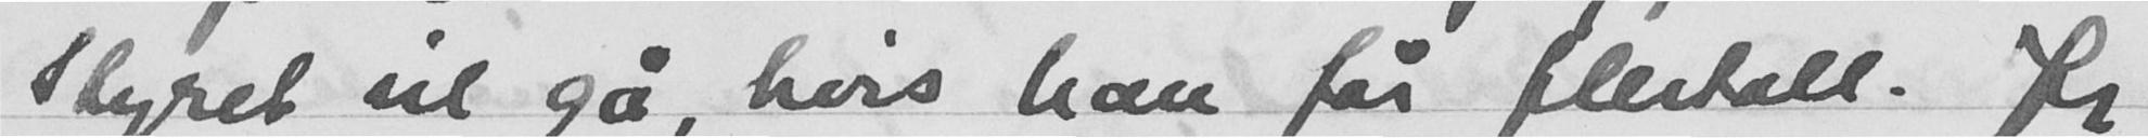Styret vil gå, hvis han får flertall. Jeg
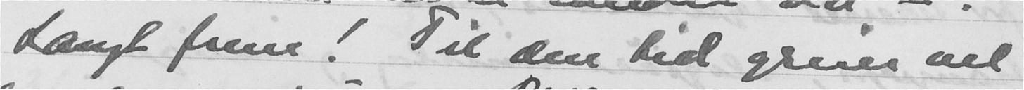Langt frem! Til den tid greier vel
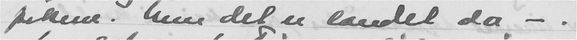pikene. Men det er landet da -.
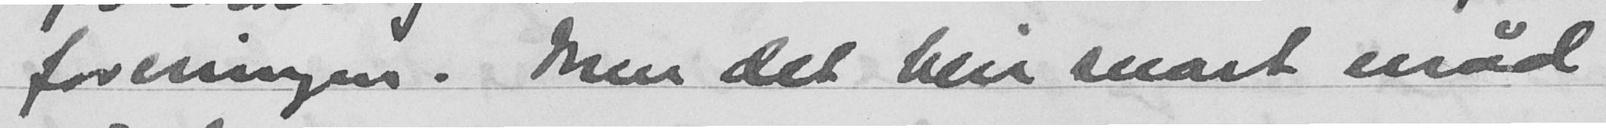foreningen. Men det blir snart nød
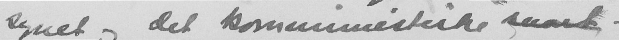synet og Det kommunistiske snart.
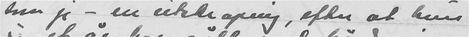som jeg - en uteke åpning, efter at hun
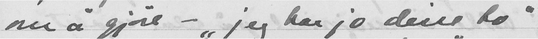om å gjøre - "jeg har jo disse to"
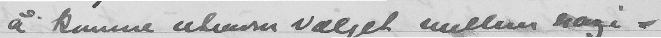å komme utenom valget mellem nagi-
